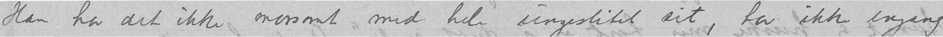Han har det ikke morsomt med hele ungeslitet sit, har ikke engang
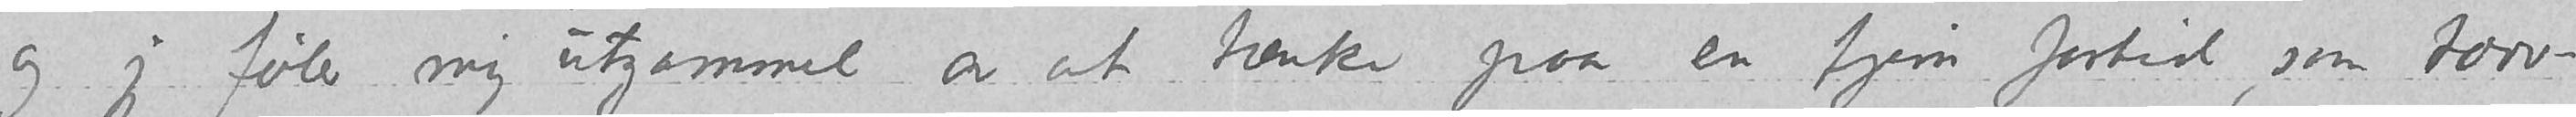og jeg føler mig utgammel av at tænke paa en fjern fortid, som torv-
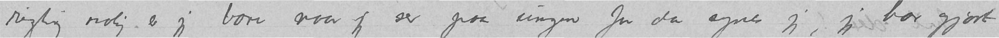rigtig rolig er jeg bare naar jeg ser paa ungen for da synes jeg, jeg har gjort
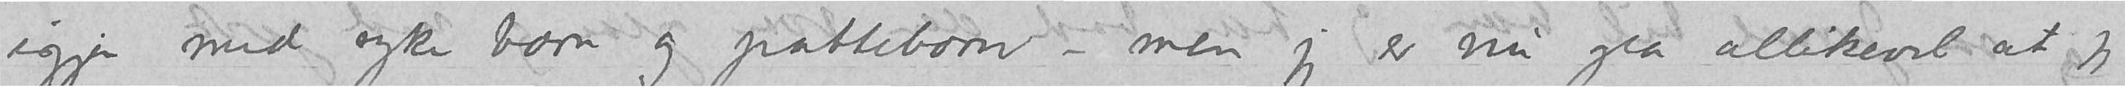igjen med syke barn og pattebarn – men jeg er nu gla allikevel at jeg
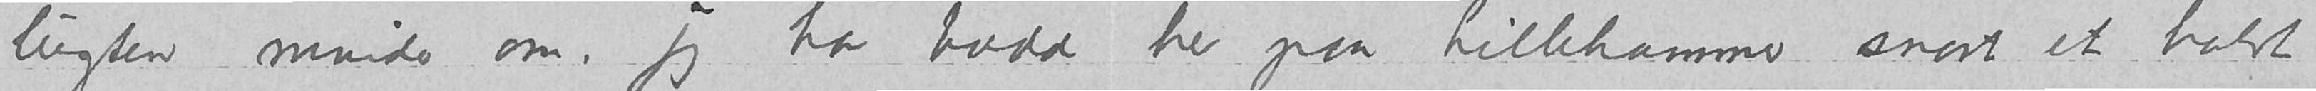lugten minder om. Jeg har bodd her paa Lillehammer snart et halvt
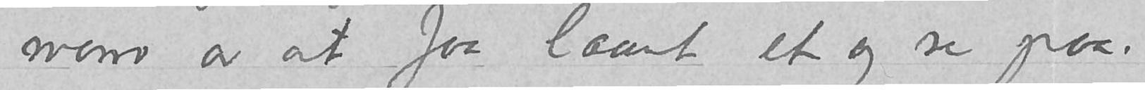morro av at faa laant et og se paa.
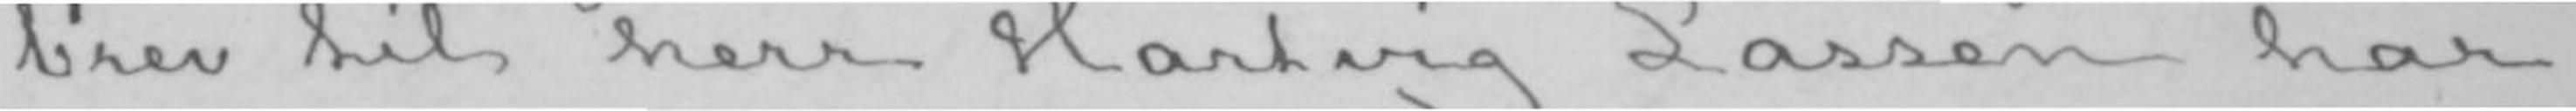brev til herr Hartvig Lassen har
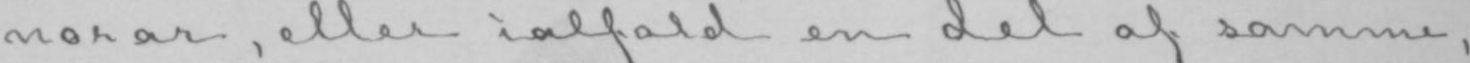norar, eller ialfald en del af samme,
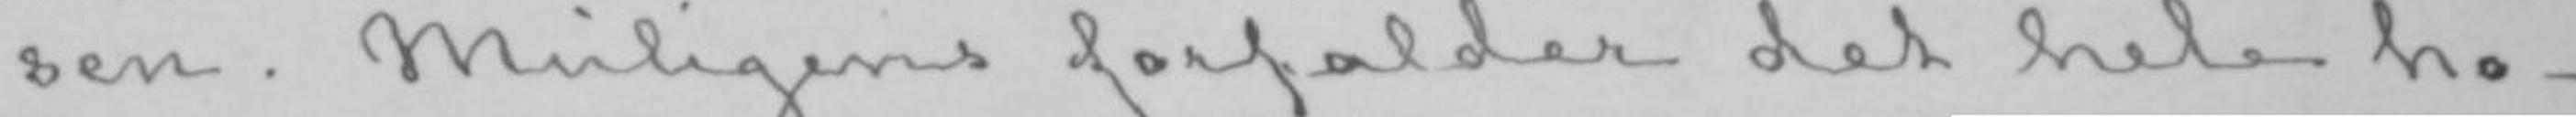sen. Muligens forfalder det hele ho-
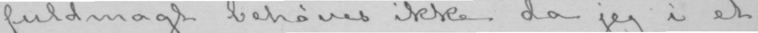fuldmagt behøves ikke da jeg i et
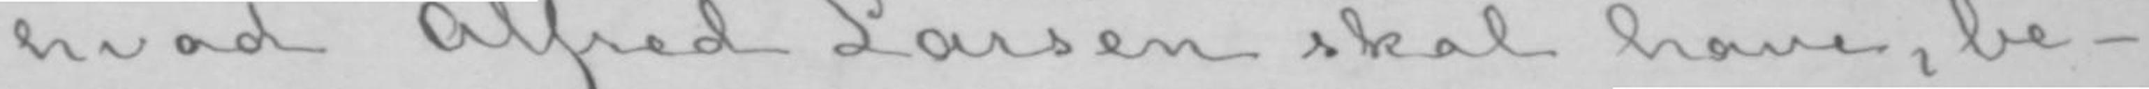hvad Alfred Larsen skal have, be-
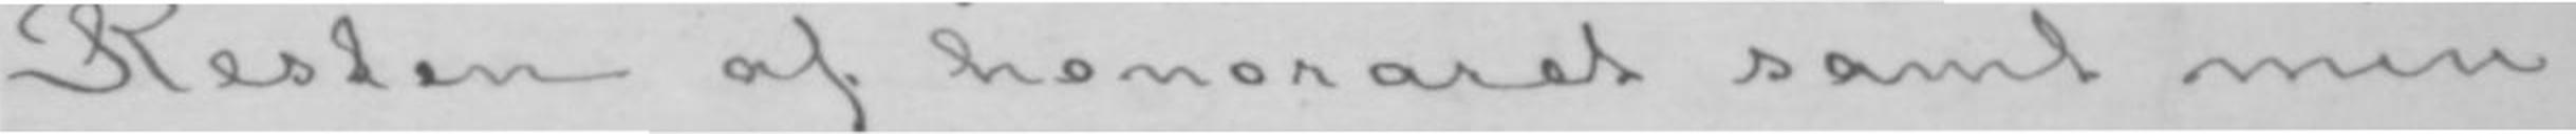Resten af honoraret samt min
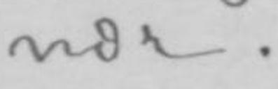nær.
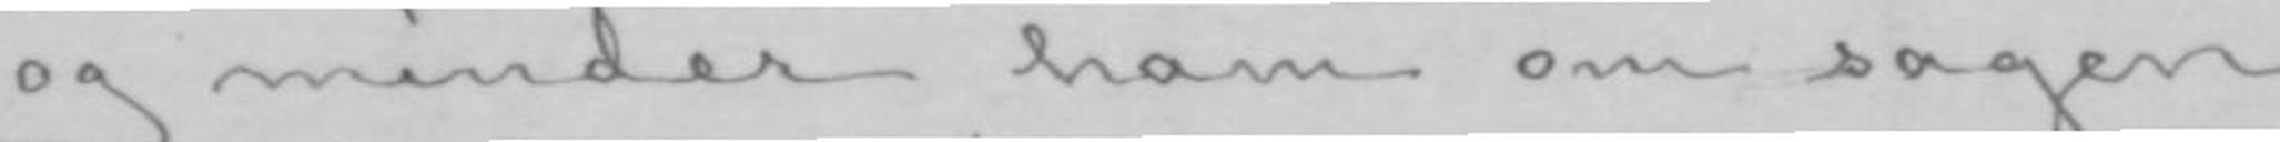og minder ham om sagen.
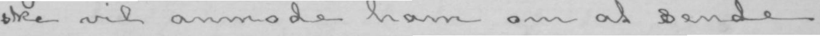ske vil anmode ham om at esende
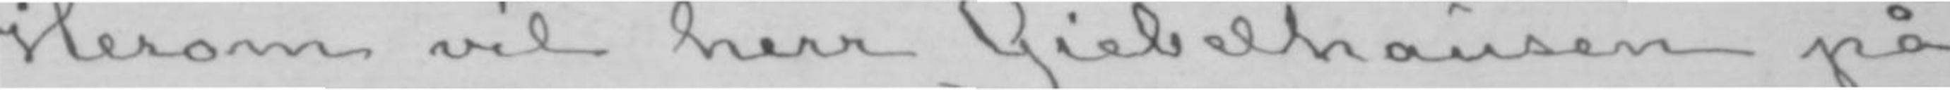Herom vil herr Giebelhausen på
